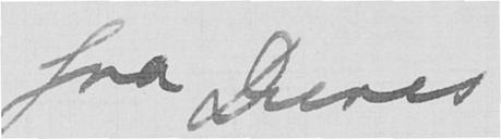fra Deres
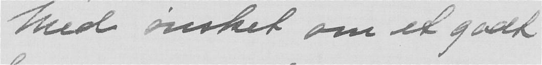Med ønsket om et godt
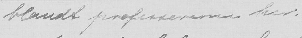blandt professorerne har.
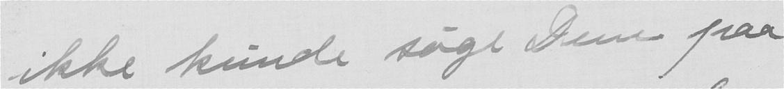ikke kunde søge Dem paa
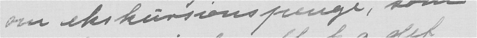om ekskursionspenge, som
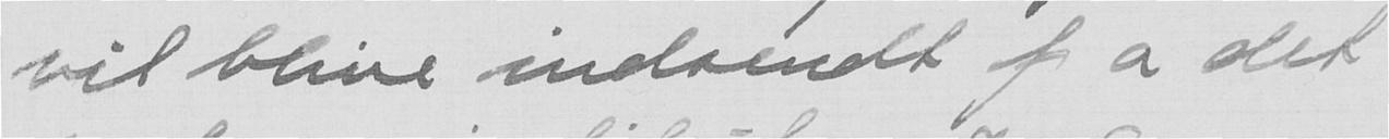vil blive indsendt fra det
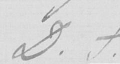D.S.
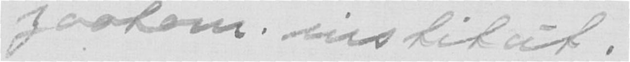zootom. institut.
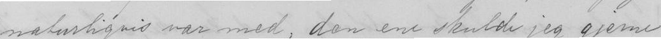naturligvis var med, den ene skulde jeg gjerne
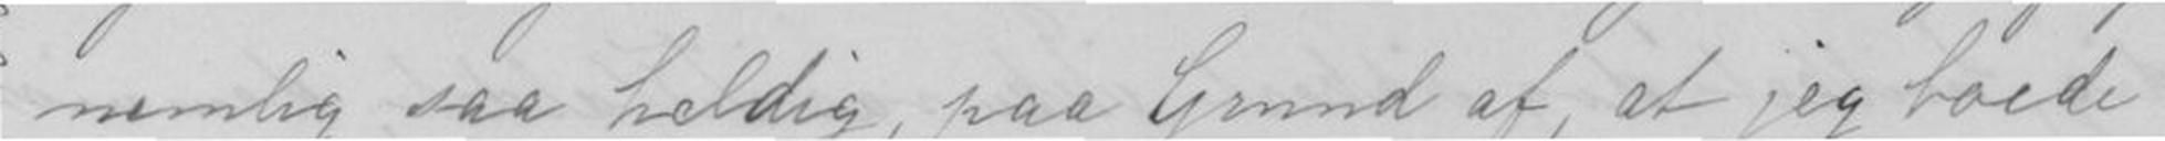nemlig saa heldig paa Grund af, at jeg lovde
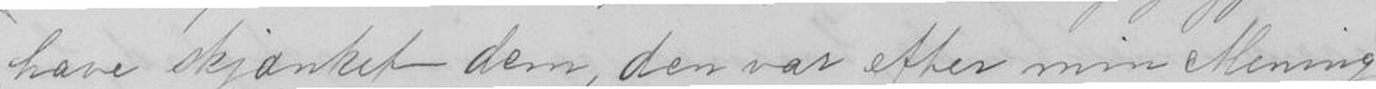have skjænket dem, den var efter min Mening
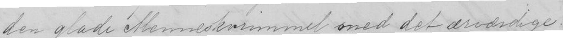den glade Menneskvrimmel med det ærværdige
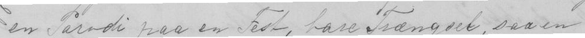en Parodi paa en Fest, have trængsel, saa en
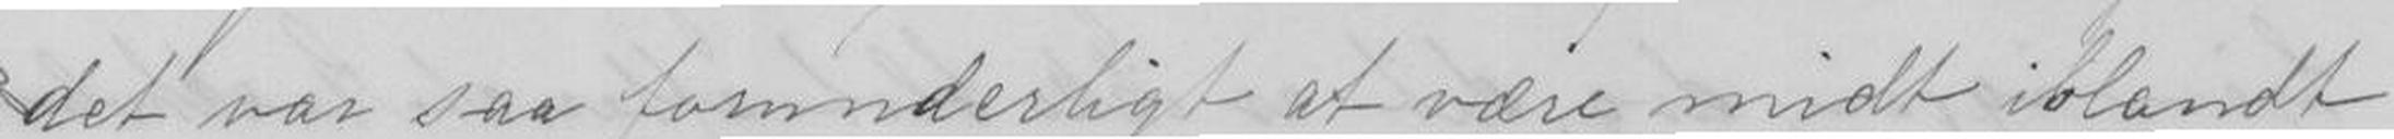det var saa forunderligt at være midt iblandt
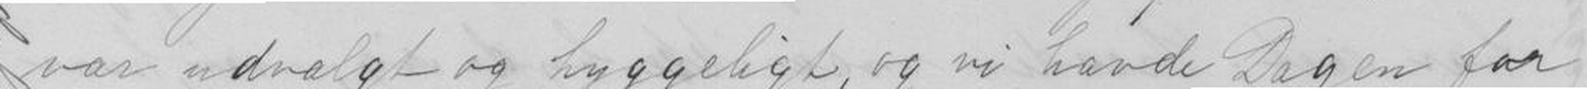var udvalgt og hyggeligt, og vi havde Dagen for
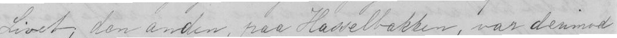Livet, den anden, paa Hasselbakken, var derimod
